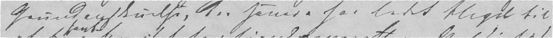Grundanskuelse, der senere har ledet Hegel til
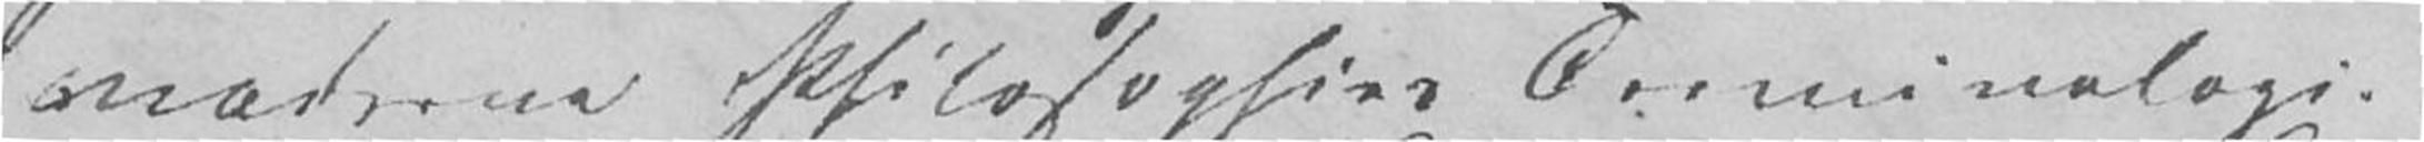moderne Philosophies Terminologi.
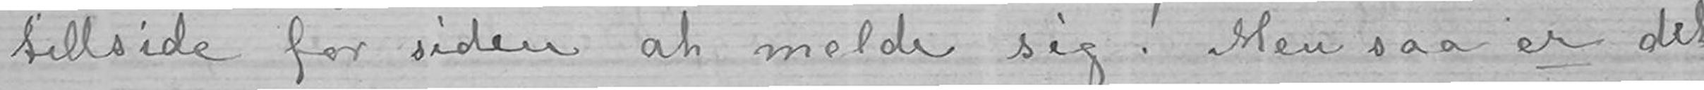tillside for siden at melde sig! Men saa er det
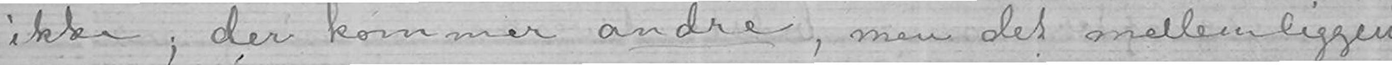ikke; der kommer andre, men det mellemliggend
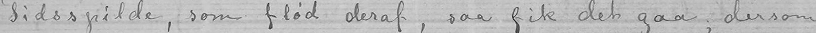Tidsspilde, som flød deraf, saa fik det gaa; dersom
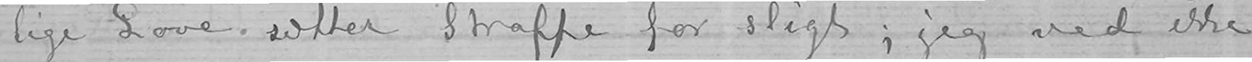lige Love sætter Straffe for sligt; jeg ved ikke
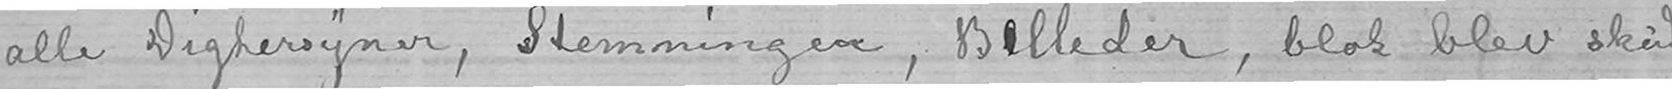alle Digtersyner, Stemninger, BillederBl, blot blev skud
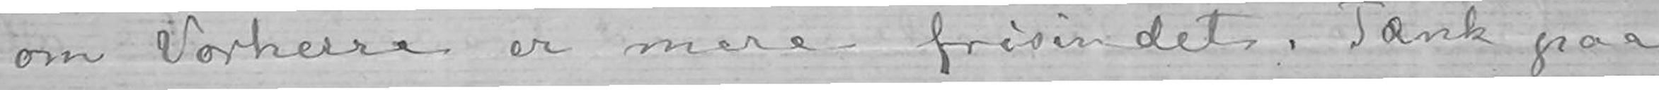om Vorherre er mere frisindet. Tænk paa
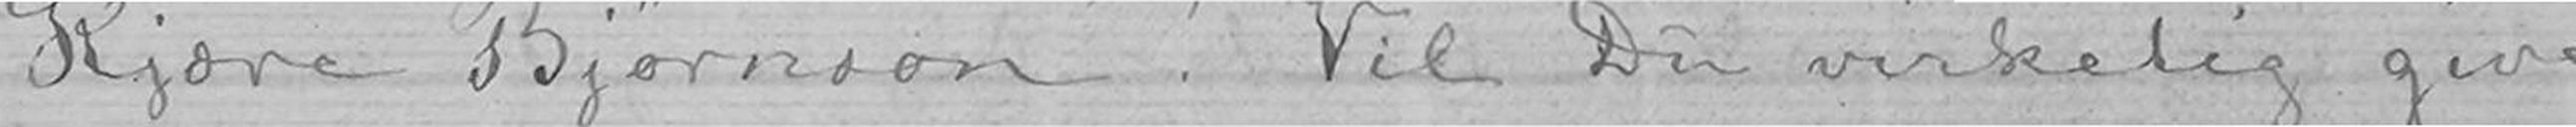Kjære Bjørnson! Vil Du virkelig give
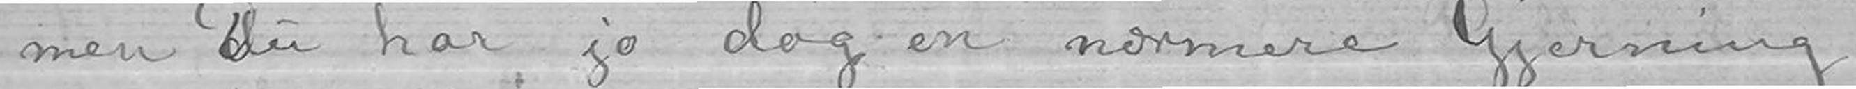men Dudu har jo dog en nærmere Gjerning
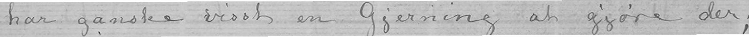har ganske visst en Gjerning at gjøre der;
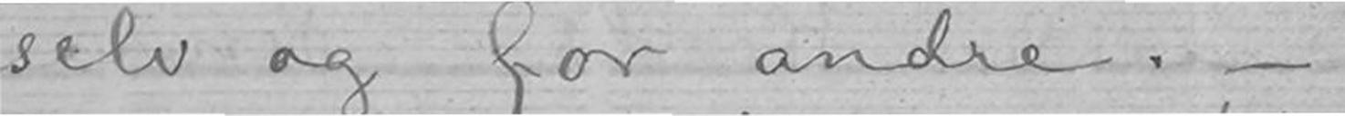selv og for andre.-
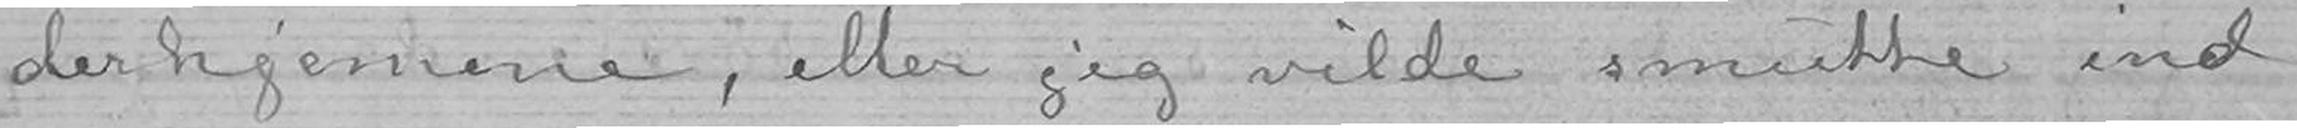derhjemme, eller jeg vilde smutte ind
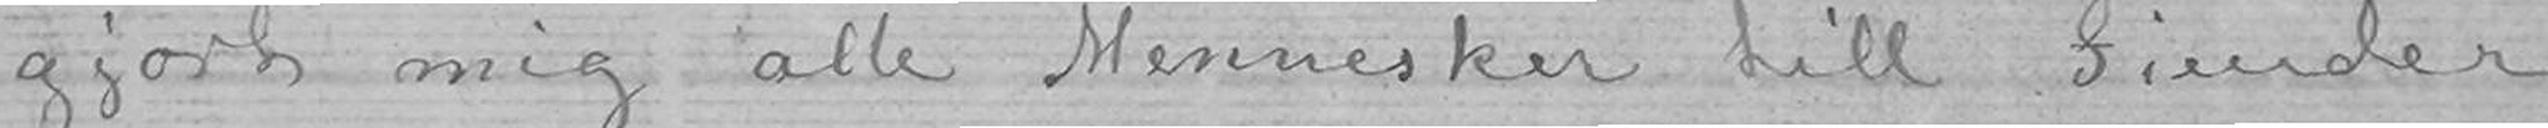gjort mig alle Mennesker till Fiender
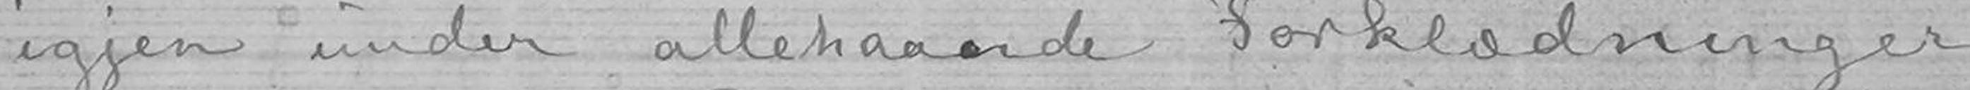igjen under allehaande Forklædninger
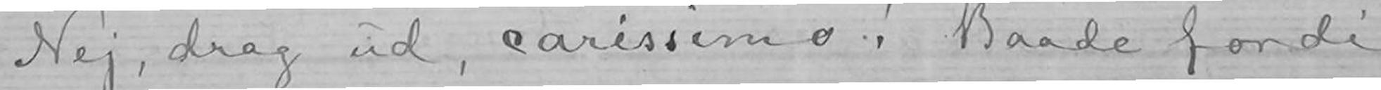Nej, drag ud, carissimo! Baade fordi
